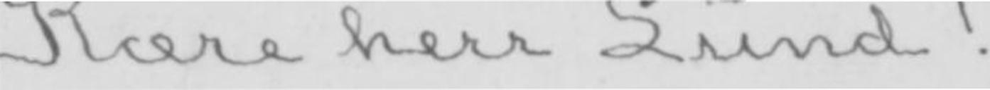Kære herr Lund!
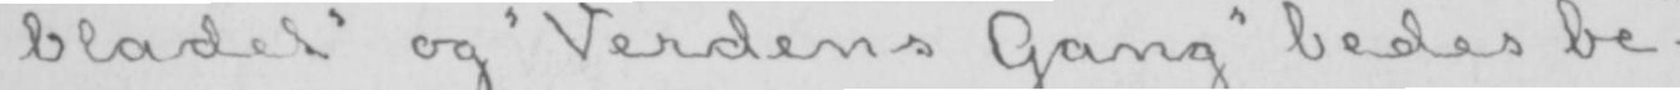bladet» og «Verdens Gang» bedes be-
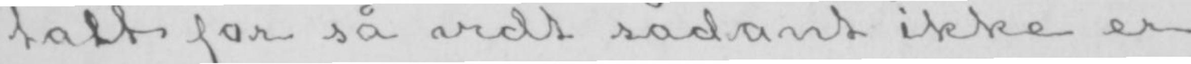talt for så vidt sådant ikke er
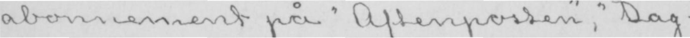abonnement på «Aftenposten», «Dag-
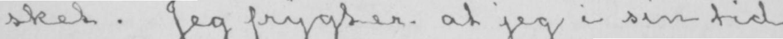sket. Jeg frygter at jeg i sin tid
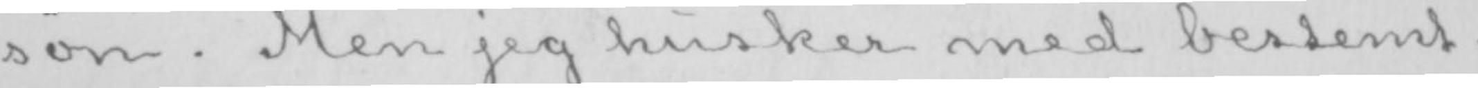søn. Men jeg husker med bestemt-
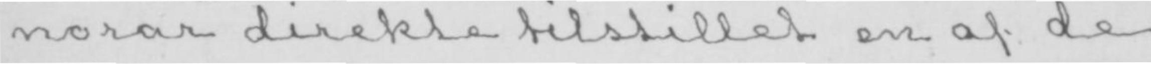norar direkte tilstillet en af de
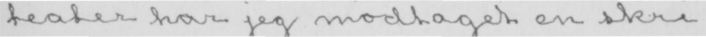teater har jeg modtaget en skri-
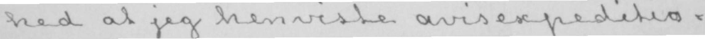hed at jeg henviste avisexpeditio-
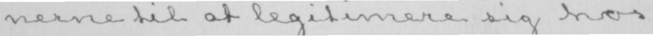nerne til at legitimere sig hos
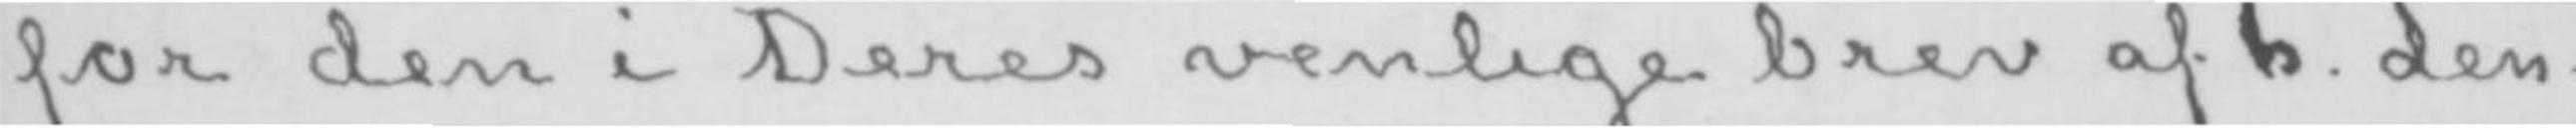for den i Deres venlige brev af 6. den-
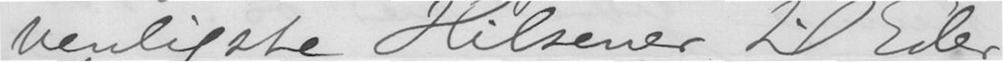venligste Hilsener til Eder
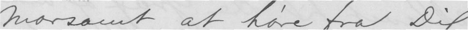morsomt at høre fra Dig
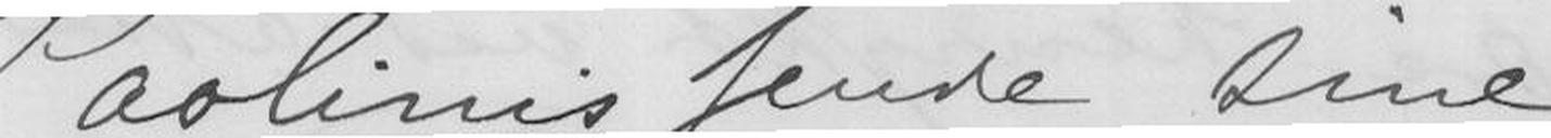Paolines sende sine
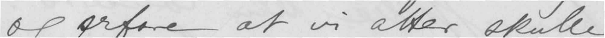og ærfare at vi atter skulle
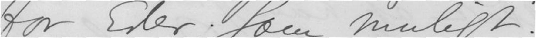for Eder som muligt.
